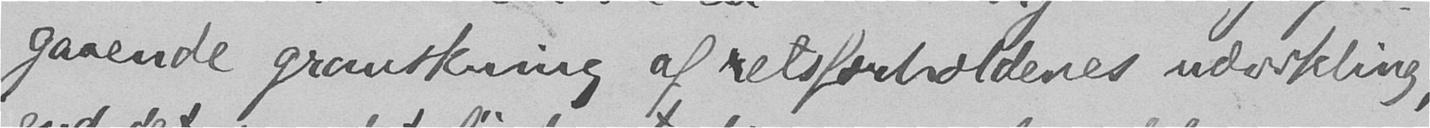gaaende granskning af retsforholdenes udvikling,
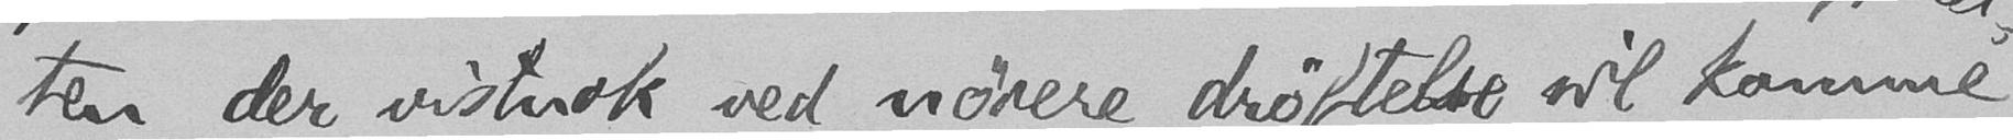ten, der vistnok ved nøiere drøftelse vil komme
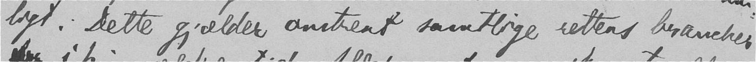ligt. Dette gjælder omtrent samtlige rettens brancher
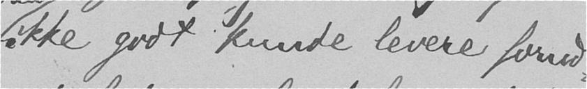ikke godt kunde levere forud-
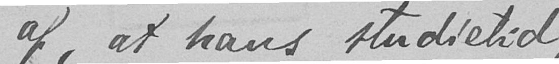af, at hans studietid
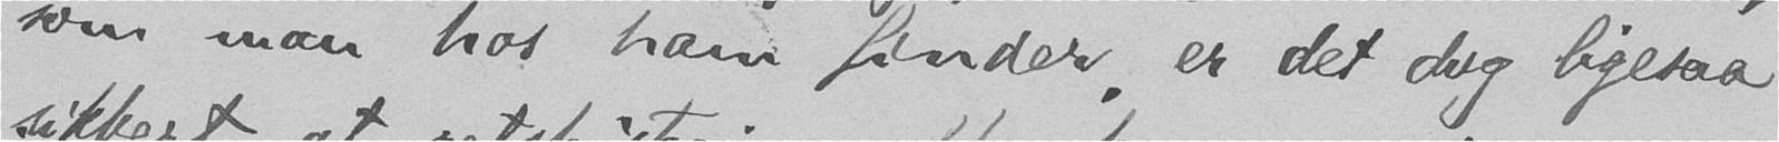som man hos ham finder, er det dog ligesaa
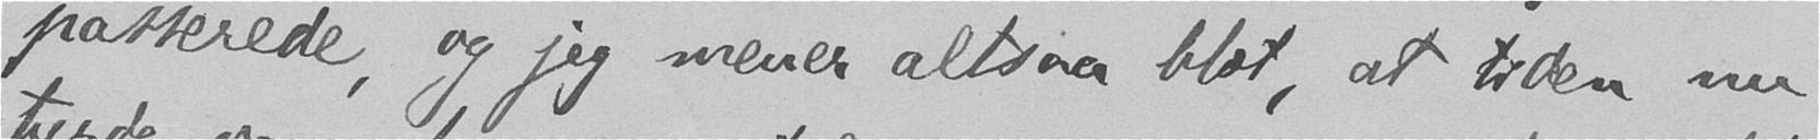passerede, og jeg mener altsaa blot at tiden nu
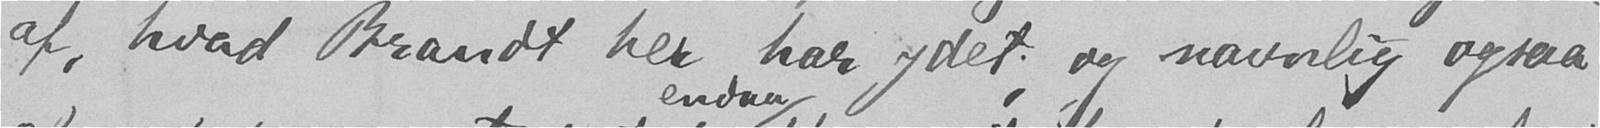af, hvad Brandt her har ydet, og navnlig ogsaa
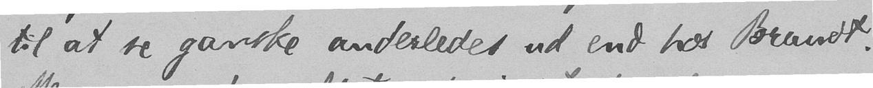til at se ganske anderledes ud end hos Brandt.
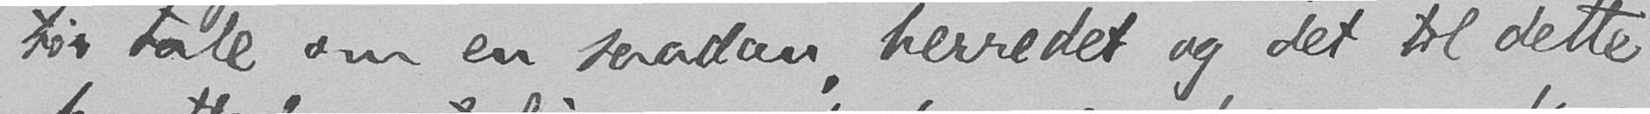tør tale om en saadan, herredet og det til dette
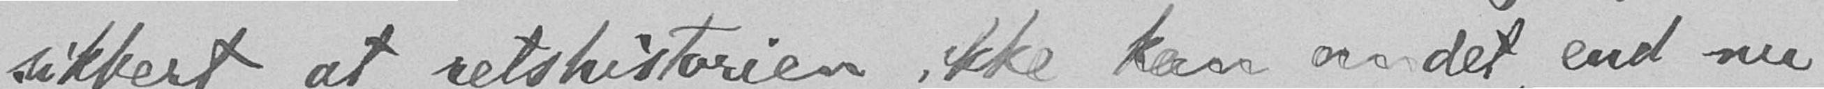sikkert, at retshistorien ikke kan andet, end nu
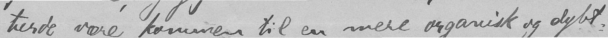turde være kommen til en mere organisk og dybt-
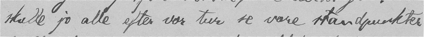skulde jo alle efter vor tur se vore standpunkter
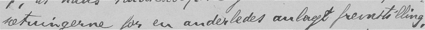sætningerne for en anderledes anlagt fremstilling,
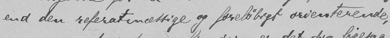end den referatmæssige og foreløbigt orienterende,
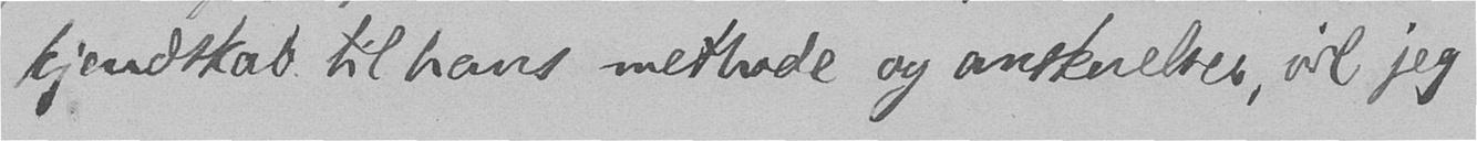kjendskab til hans methode og anskuelser, vil jeg
